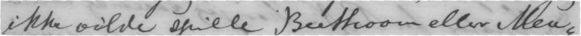ikke vilde spille Beethoven eller Men-
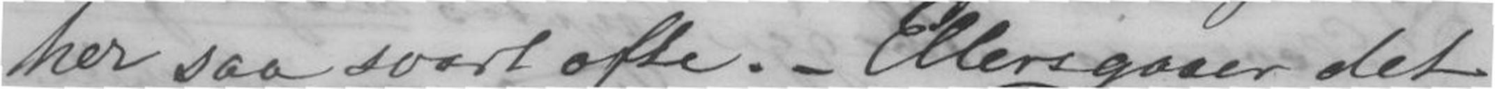her saa svært ofte. - Ellersgaaer det
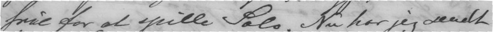frie for at spille Solo. Nu har jeg sendt
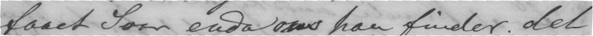faaet Svar enda om han finder det
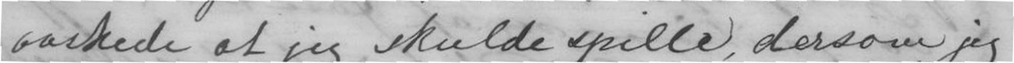ønskede at jeg skulde spille dersom jeg
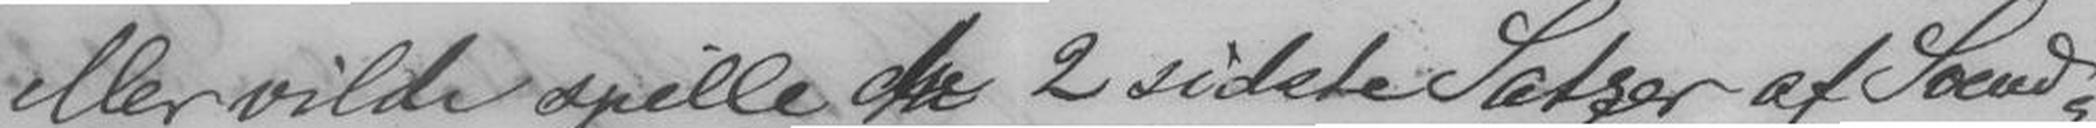eller vilde spille de 2 sidste Satzer af Svend-
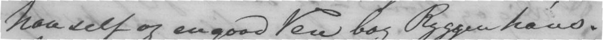han self og en good Ven bag Ryggen hans.
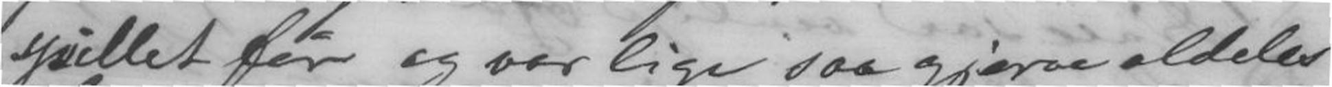spillet før og var lige saa gjerne aldeles
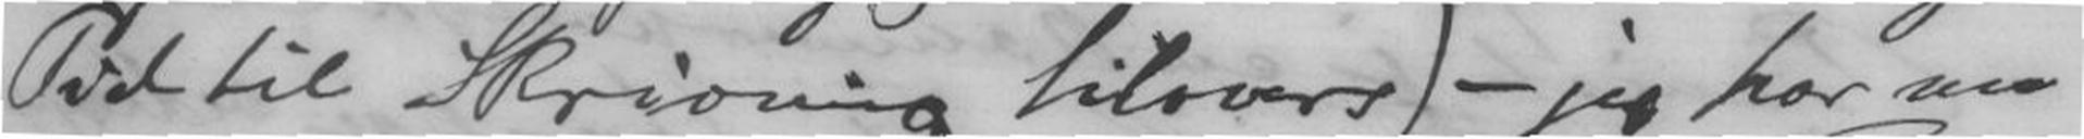Tid til Skrivning tilovers) - jeg har nu
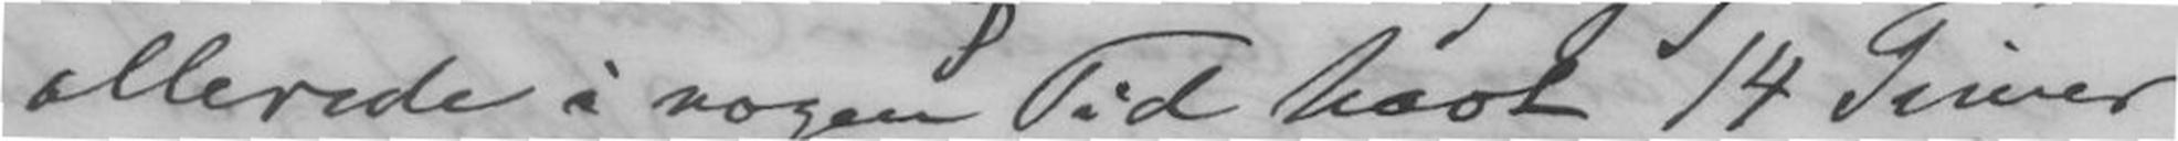allerede i nogen Tid havt 14 Timer
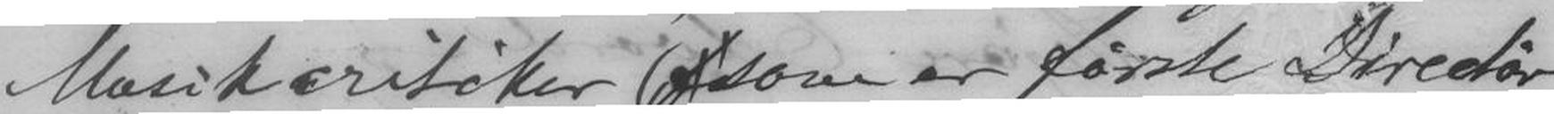Musik aritiker (som er første Direktør
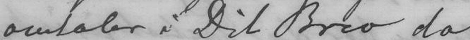omtaler i Dit Brev da
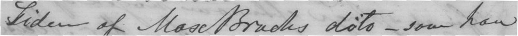Siden af Mas Brades døto - som han
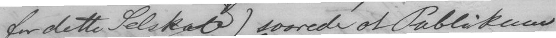for dette Selskab) svarede at Publikum
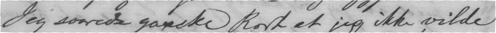Jeg svarede ganske kort at jeg ikke vilde
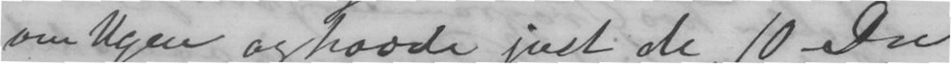om Ugen og havde just de 10 Du
